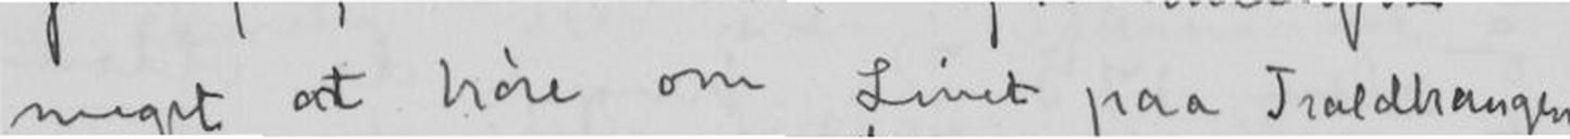meget at høre om Livet paa Troldhaugen
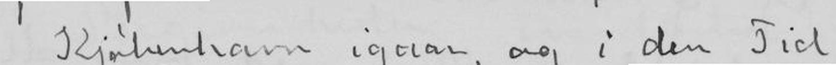i Kjøbenhavn igaar, og i den Tid
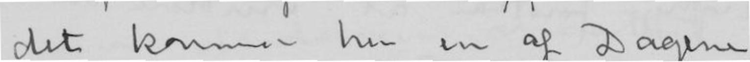det kommer er en af Dagene,
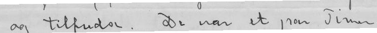og tilfredse. De var et par Timer
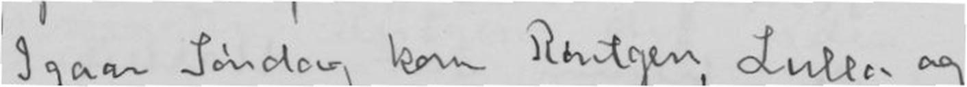Igaar Søndag kom Röntgen, Lullo, og
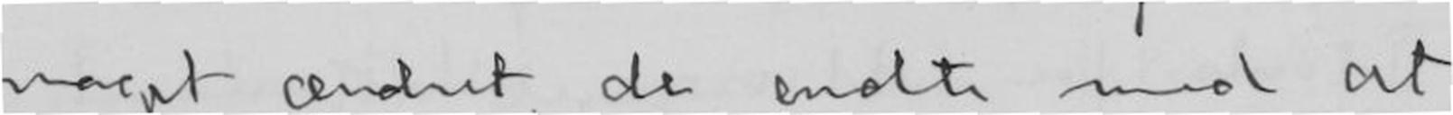noget andet, de endte med at
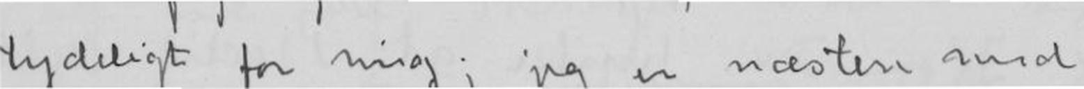tydeligt for mig; jeg er næsten med
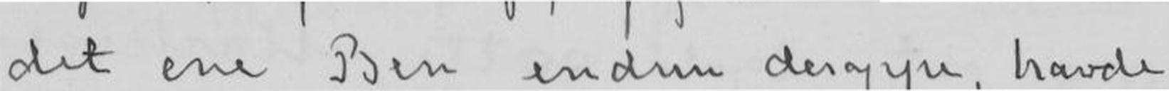det ene Ben endun deroppe, havde
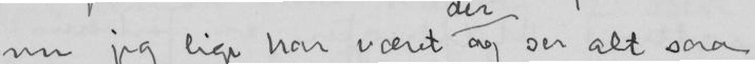nu jeg lige har været og ser alt saa
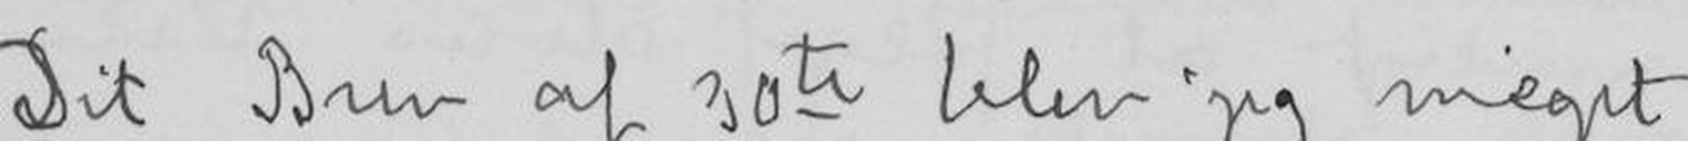Dit Brev af 30te blev jeg meget
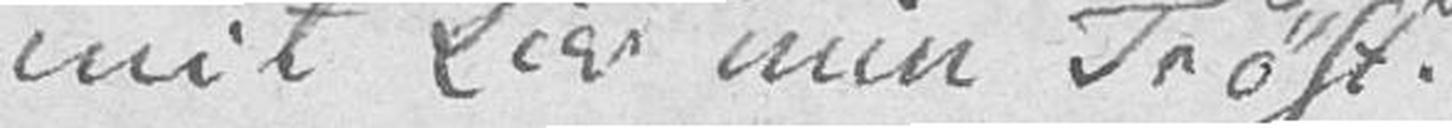mit Liv min Trøst.
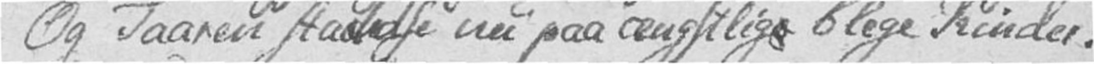Og Taaren standse nu på ængstlige blege Kinder!
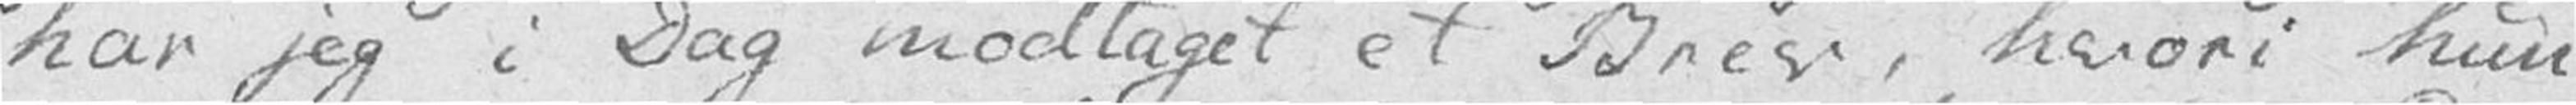har jeg i Dag modtaget et Brev, hvori hun
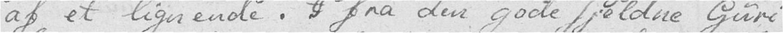af et lignende. I fra den gode sjeldne Guri
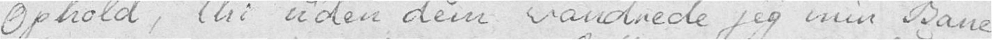Ophold, thi uden dem vandrede jeg min Bane
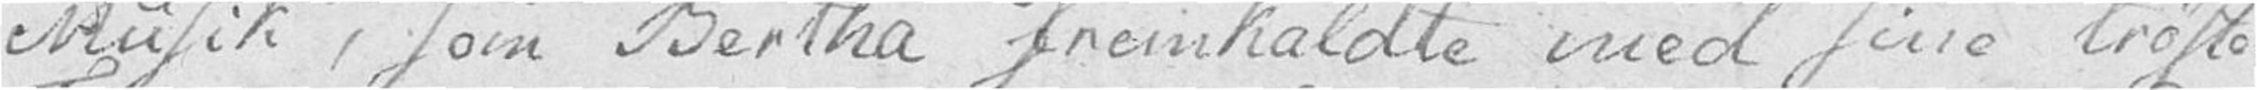Musik, som Bertha fremkaldte med sine trøste
